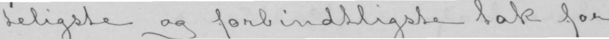teligste og forbindtligste tak for
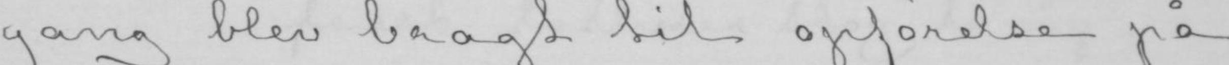gang blev bragt til opførelse på
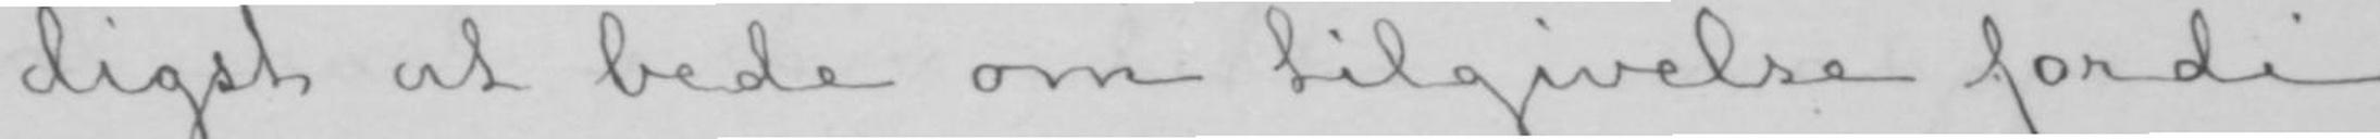digst at bede om tilgivelse fordi
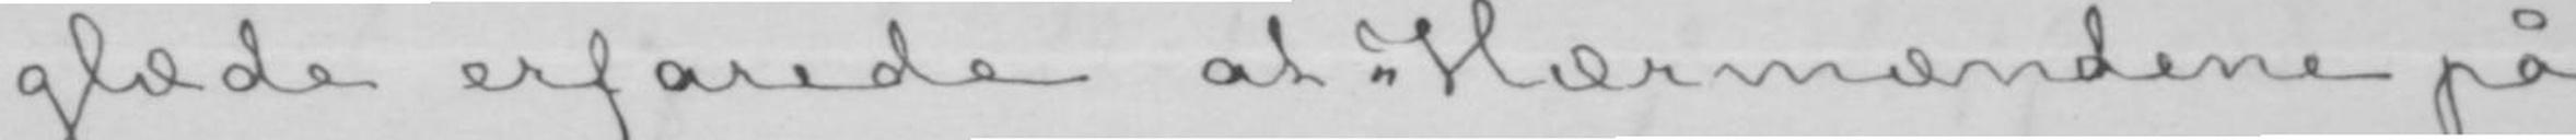glæde erfarede at «Hærmændene på
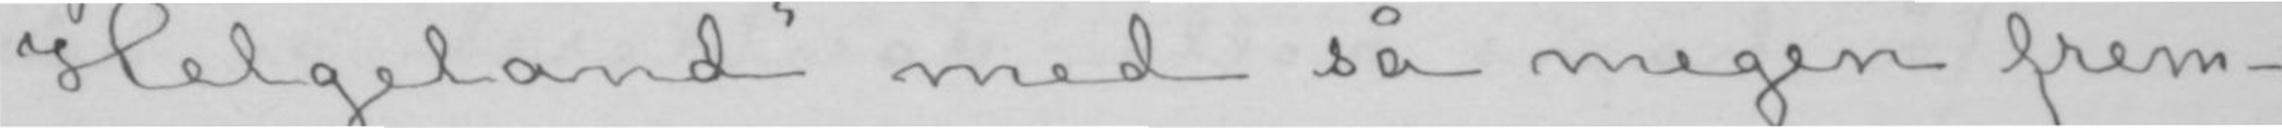Helgeland» med så megen frem-
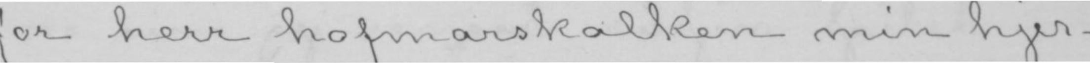for herr hofmarskalken min hjer-
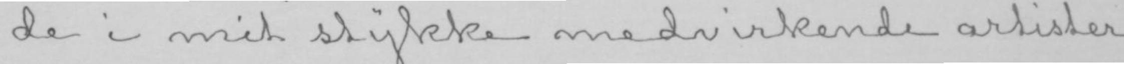de i mit stykke medvirkende artister
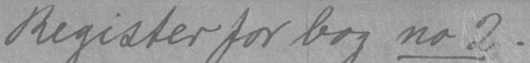Register for bog no 2.
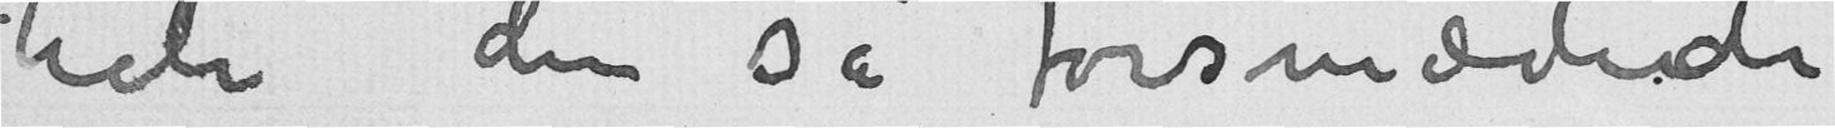hele den så forsmædede
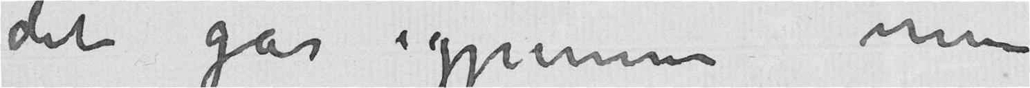det går igjennem men
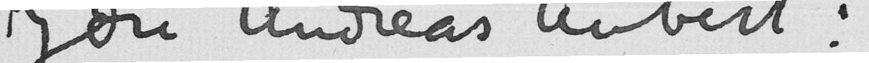Kjære Andreas Aubert!
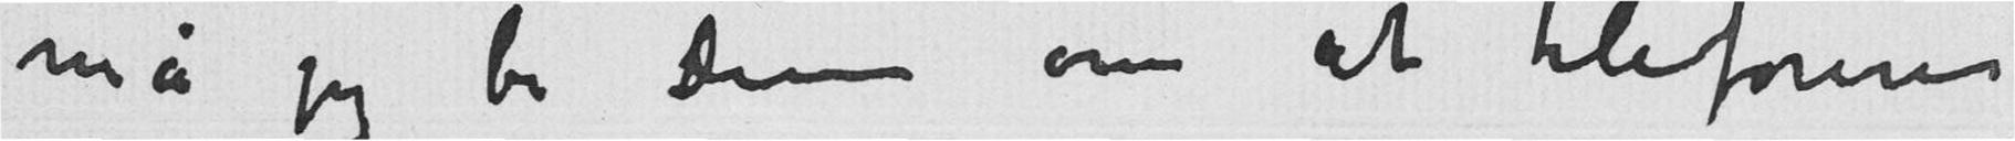må jeg be Dem om at telefonere
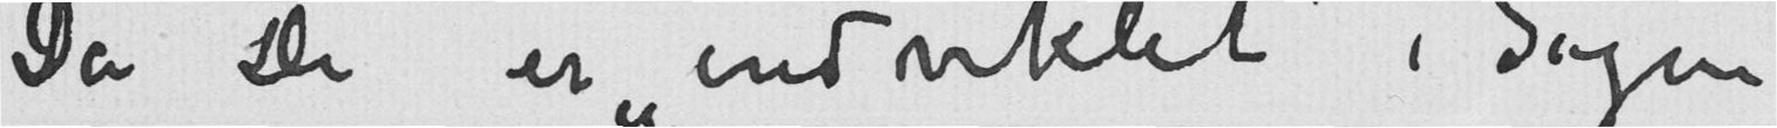Da De er «indviklet» i Sagen
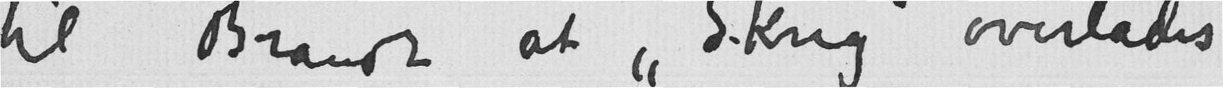til Brandt at «Skrig» overlades
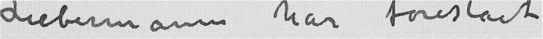Liebermann har foreslået
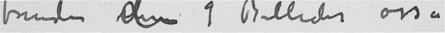foruden de 9 Billeder osså
